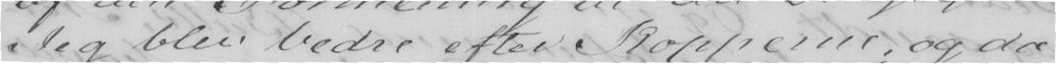Jeg blev bedre efter Kopperne, og da
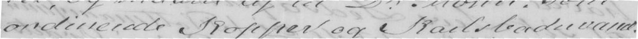ordinerede Kopper og Karlsbadervand.
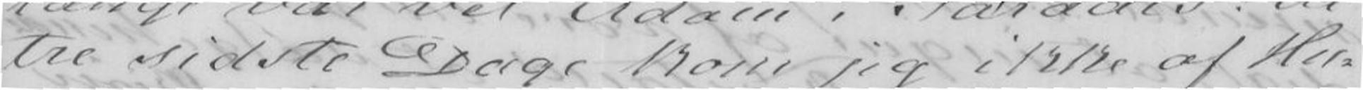tre sidste Dage kom jeg ikke af Hu-
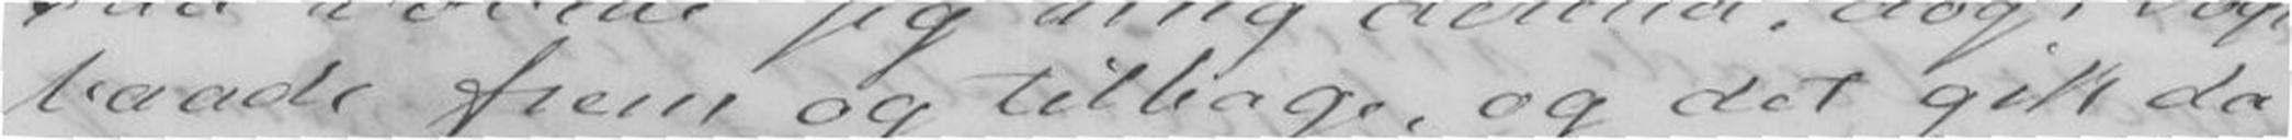baade frem og tilbage, og det gik da
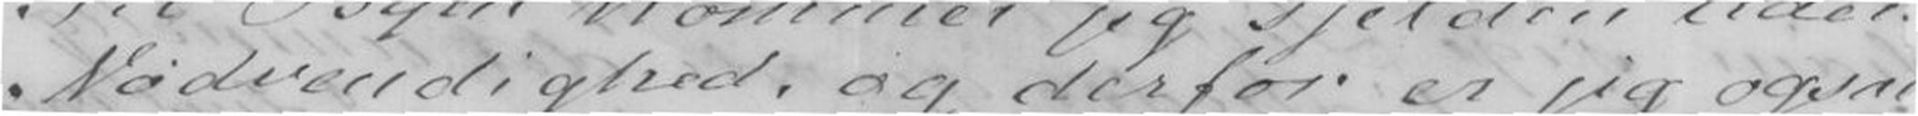Nødvendighed, og derfør er jeg ogsa
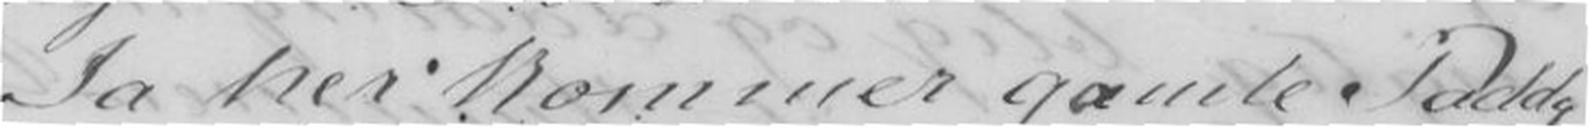Ja her kommer gamle Paddy,
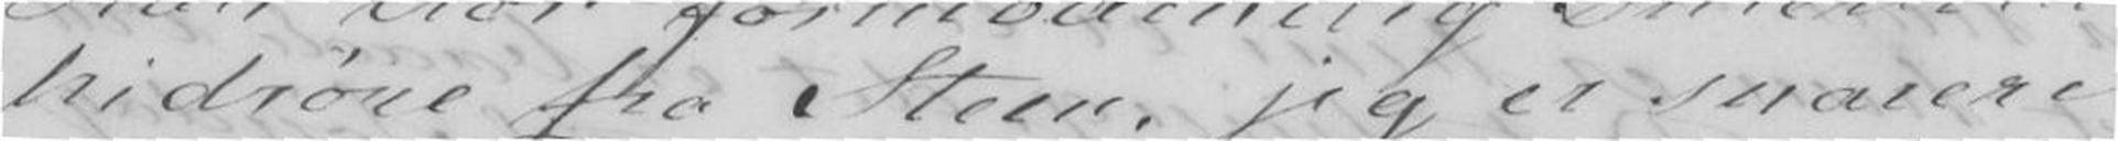hidrøne fra Steen, jeg er snarere
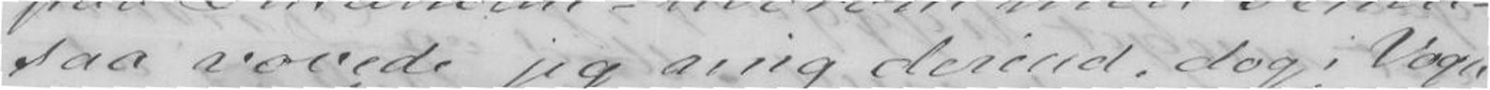saa vovede jeg mig derind og i Vogn
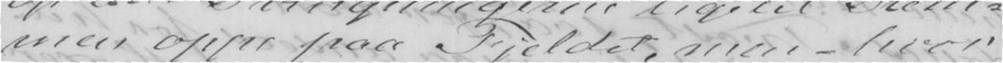men oppe paa Fjeldet, men hvor
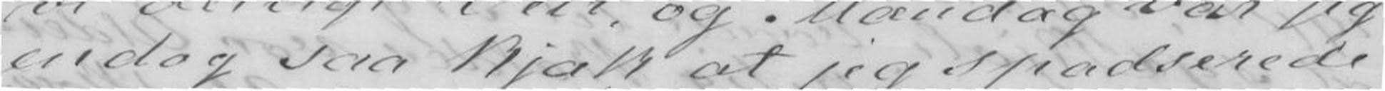endog saa kjæk at jeg spadserede
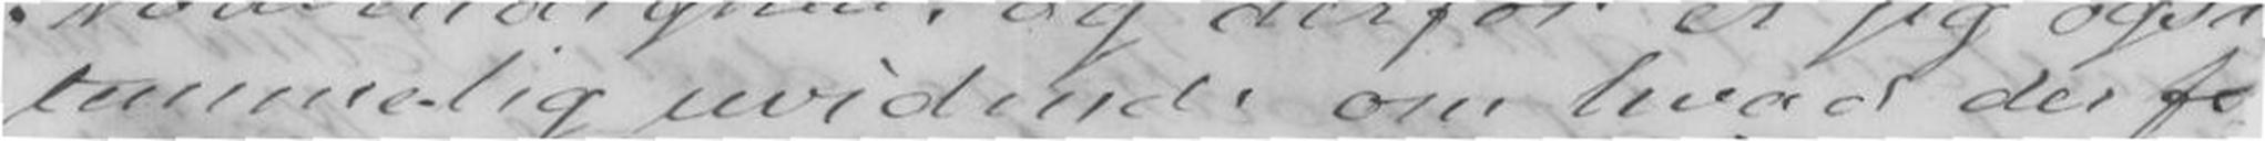temmelig uvidende om hvad der fo
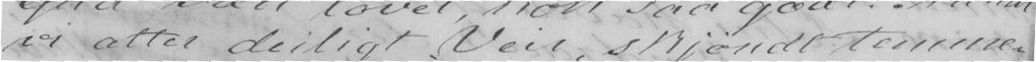vi atter deiligt Veir, skjøndt temme-
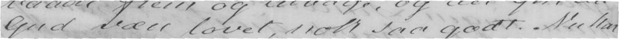Gud være lovet, nok saa godt. Nu har
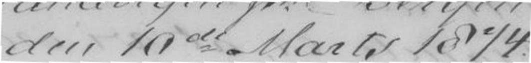den 10de Marts 1874.
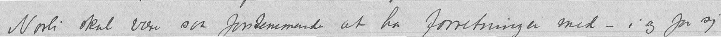Norli skal være saa forstemmende at ha forretninger med – i og for sig
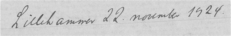Lillehammer 22. november 1924.
